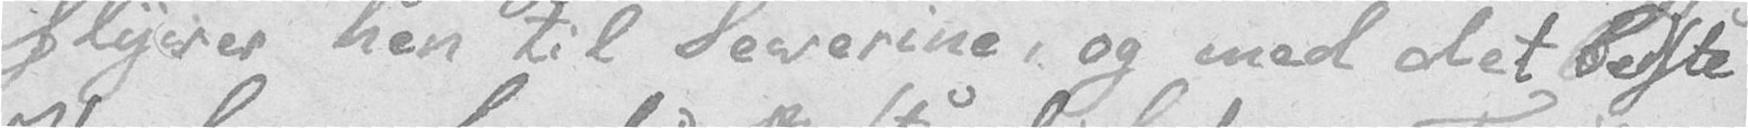flyver hen til Severine, og med det bedste
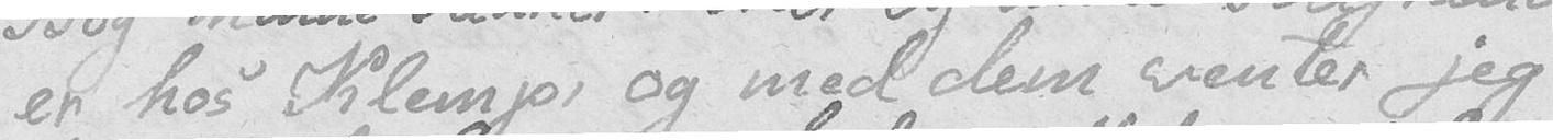er hos Klemp, og med dem venter jeg
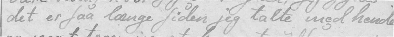det er saa længe siden jeg talte med hende
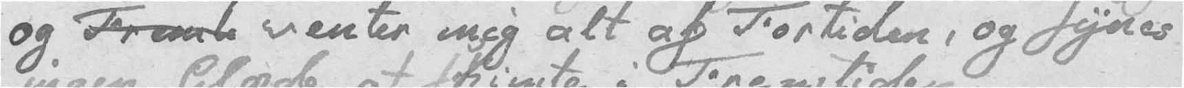og Fremle venter mig alt af Fortiden, og synes
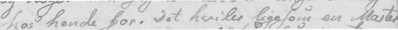hos hende for. Det hviler ligesom en Marter
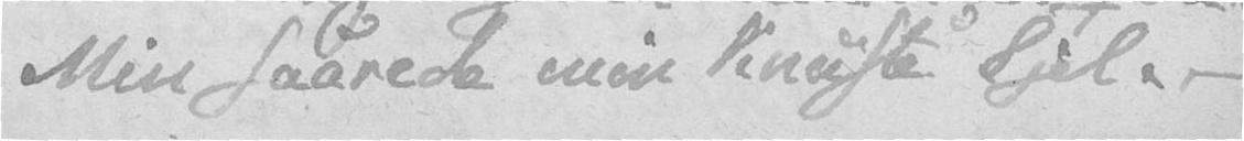Min saarede min knuste Sjel. –
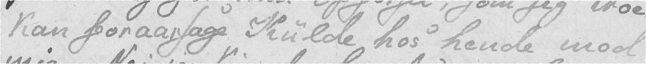kan foraarsage Kulde hos hende mod
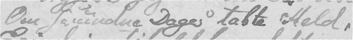Om svundne Dages tabte Held,
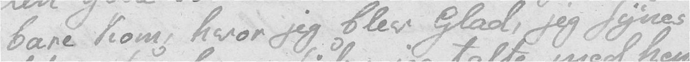bare kom, hvor jeg blev Glad, jeg synes
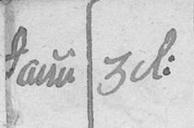Janu 3d:
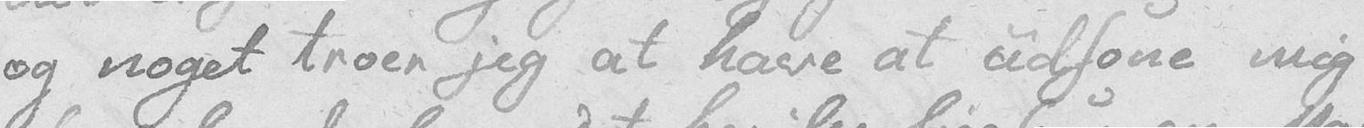og noget troer jeg at have at udsone mig
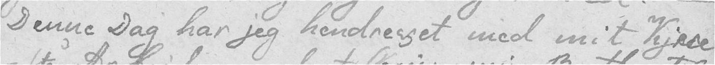Denne Dag har jeg hendrevet med mit Kjere
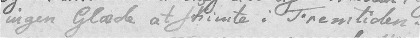ingen Glæde at skimte i Fremtiden. –
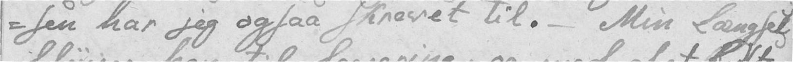-sen har jeg ogsaa skrevet til. – Min Længsel
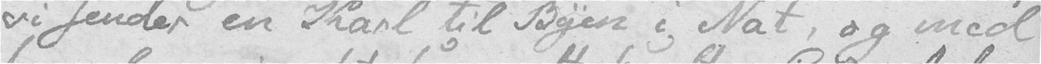vi sender en Karl til Byen i Nat, og med
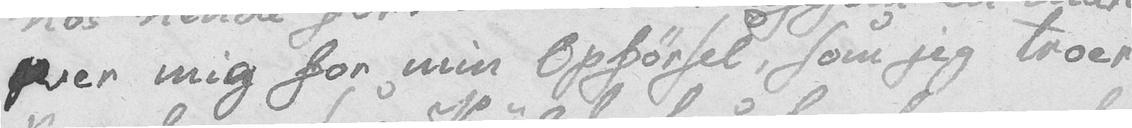over mig for min Opførsel, som jeg troer
